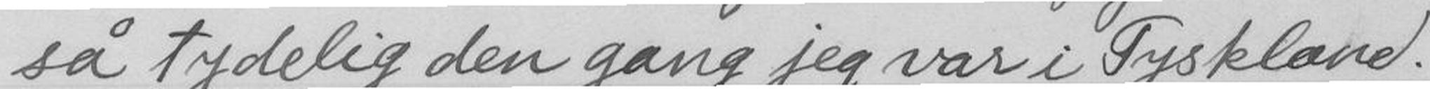så tydelig den gang jeg var i Tyskland.
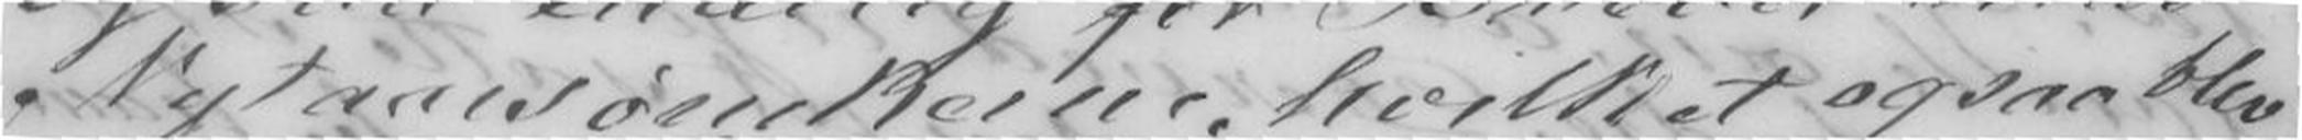Nytaarsønskerne, hvilket ogsaa blev
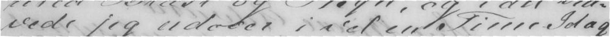vede jeg udover i vel en Time. Idag
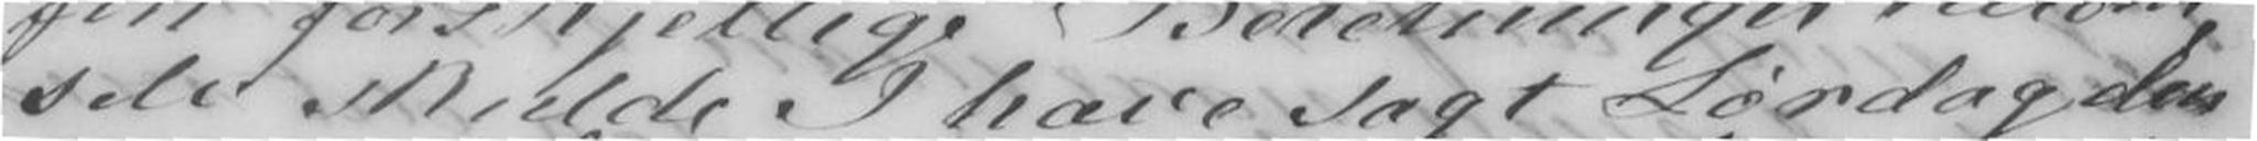selv skulde I have sagt Lørdag den
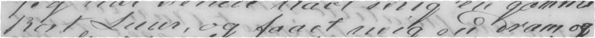kort Luur, og faaet mig en Dram og
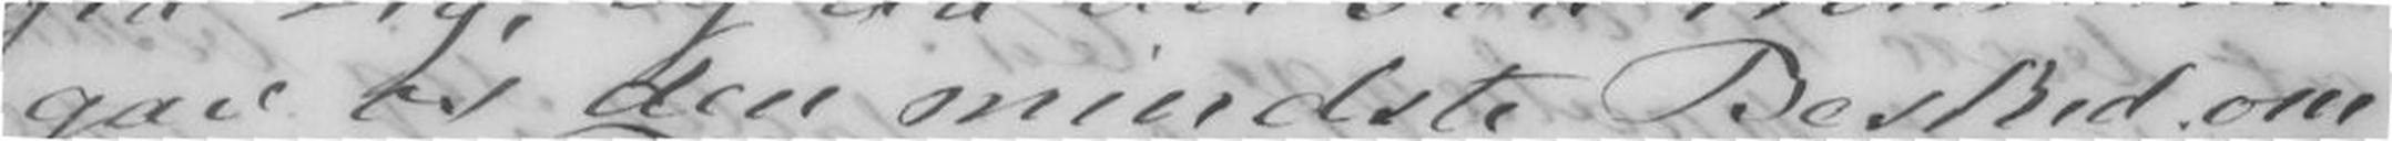gav os den mindste Besked om
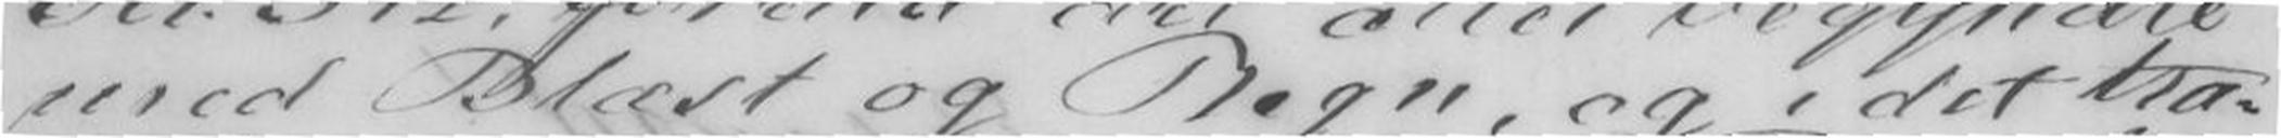med Blæst og Regn, og i det tra-
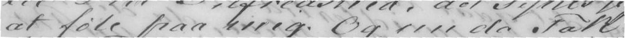at føle paa mig. Og nu da Tak
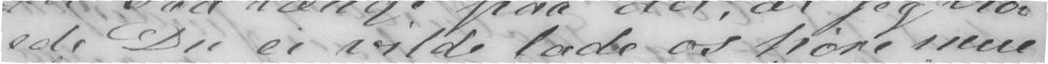ede Du ei vilde lade os høre mere
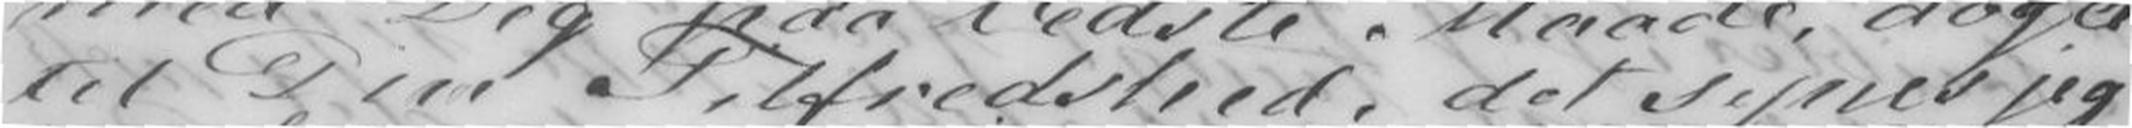til Din Tilfredshed, det synes jeg
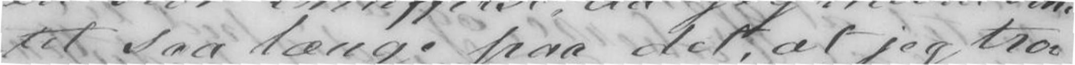tet saa længe paa det, at jeg tro-
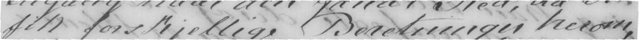fik forskjellige Beretninger herom
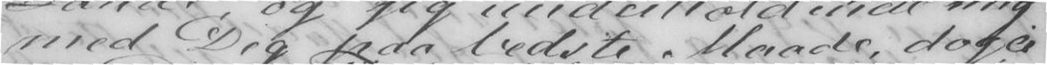med Dig paa bedste Maade dog ei
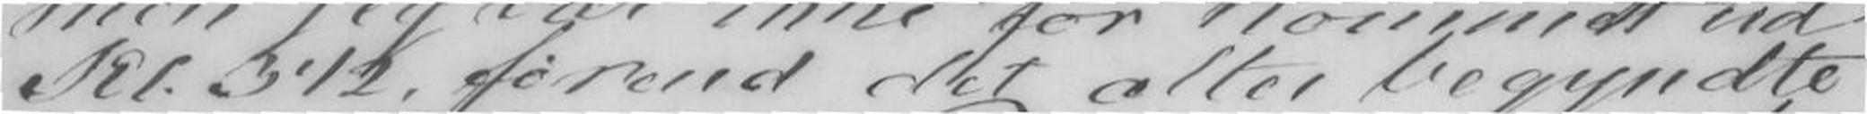Kl. 3½, førend det atter begyndte
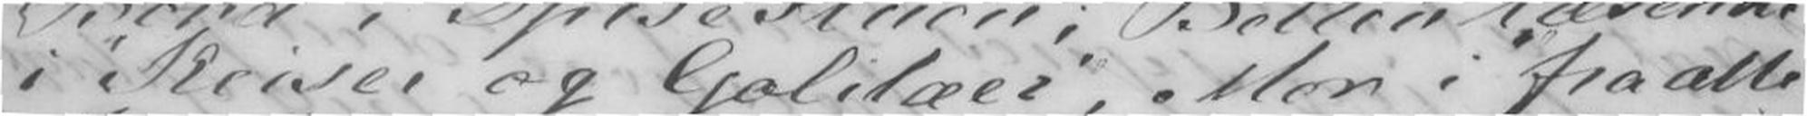i "Keiser og Galilæer. Men i fra alle
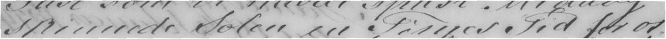skinnede solen en Times Tid for os,
